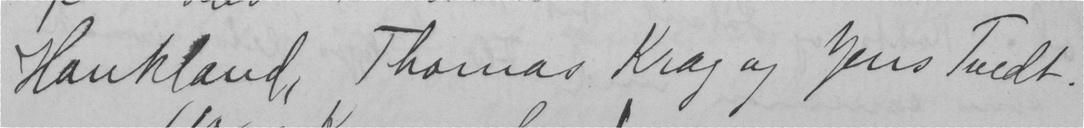Haukland, Thomas Krag og Jens Tvedt.
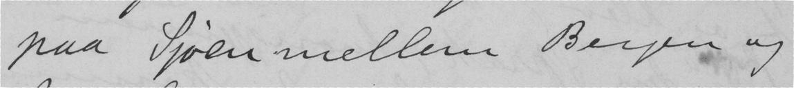paa Sjøen mellem Bergen og
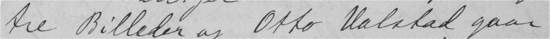tre Billeder og Otto Valstad gaar
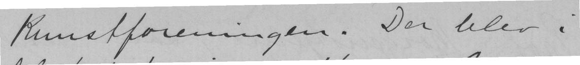Kunstforeningen. Der blev i
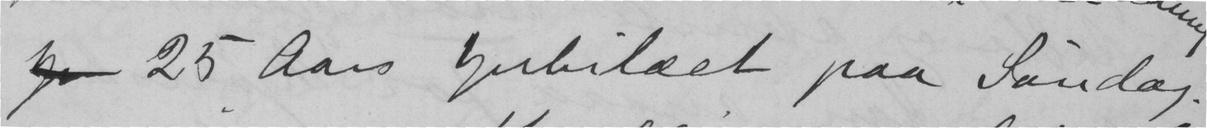25 Aars Jubilæet paa Søndag.
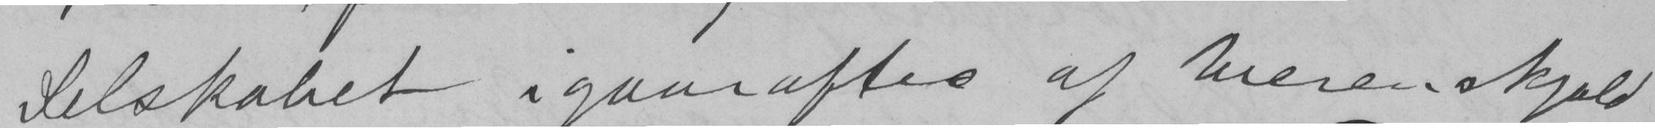Selskabet igaaraftes af Werenskjold.
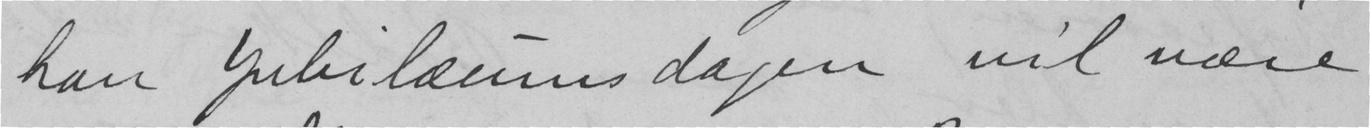han Jubilæumsdagen vil være
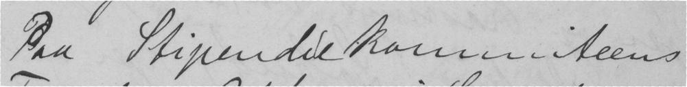Paa Stipendiekommiteens
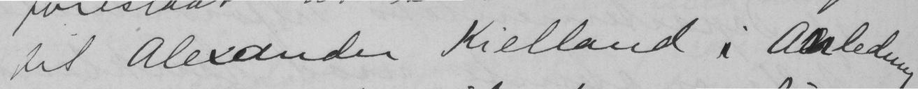til Alexander Kielland i Ansledning
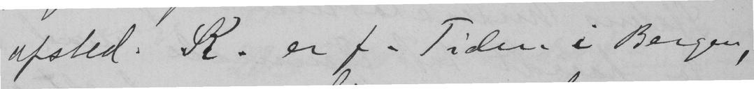afsted. K. er f. Tiden i Bergen,
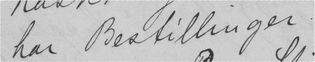har Bestillinger.
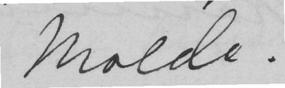Molde.
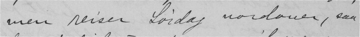men reiser Lørdag nordover, saa
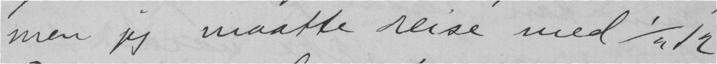men jeg maatte reise med 1/2 12
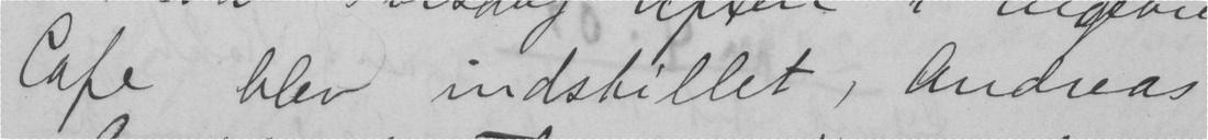Cafe blev indstillet, Andreas
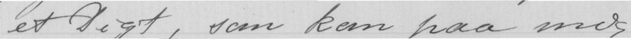et Digt, som kom paa meg
